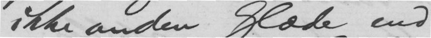"ikke anden Glæde end
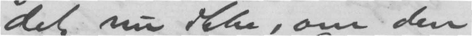det nu ikke, om den
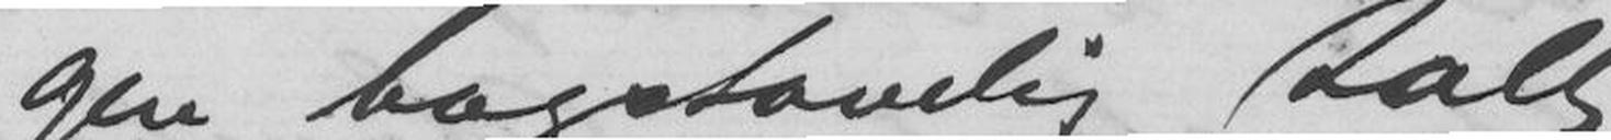gen bogstavelig talt
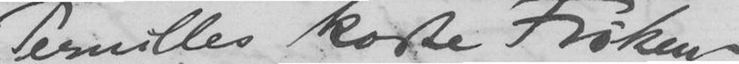Pernilles korte Frøken-
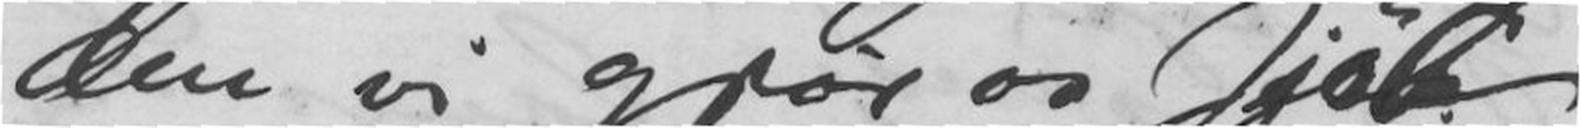den vi gjør os sjøl".
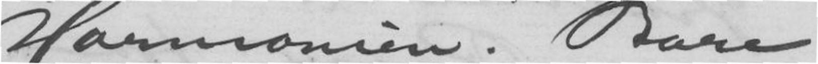"Harmonien". Bare
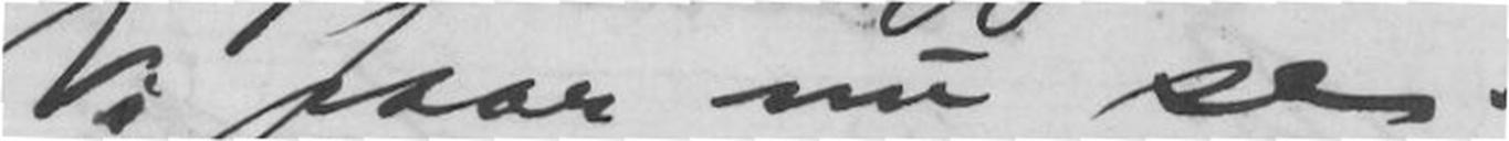Vi faar nu se.
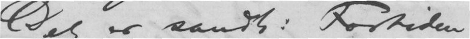Det er sandt: Fortiden
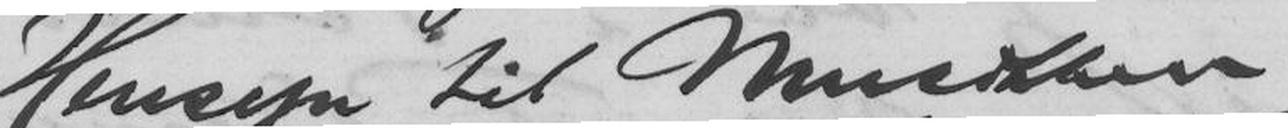Hensyn til Musikken
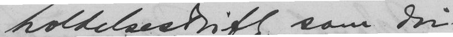holdelsesdrift som dri
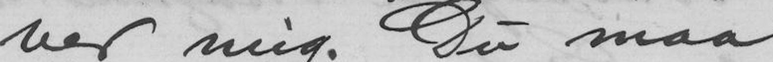- ver mig. Du maa
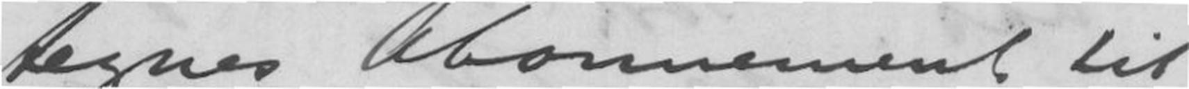tegnes Abonnement til
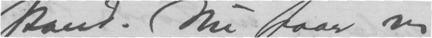stand. Nu faar vi
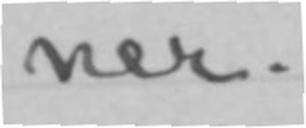ner.
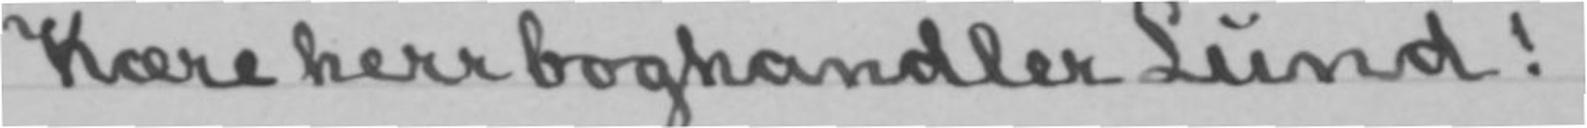Kære herr boghandler Lund!
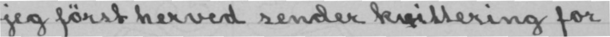jeg først herved sender kvittering for
